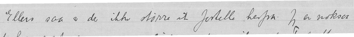Ellers saa er der ikke større at fortelle herfra. Jeg er noksaa
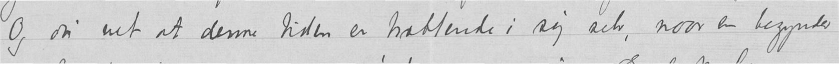Og du vet at denne tiden er trættende i sig selv, naar en begynder
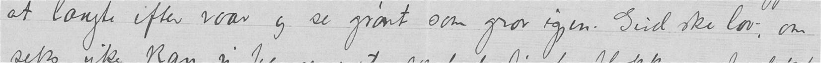at længte efter vaar og se grønt som gror igjen. Gud ske lov, om
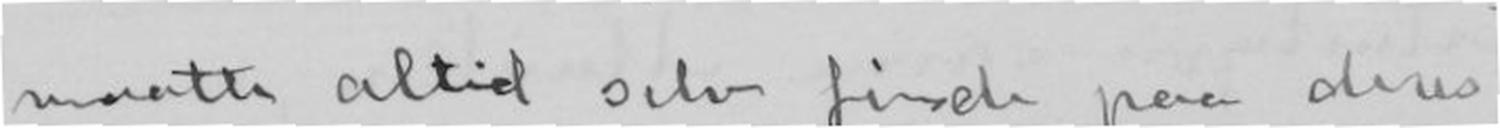maate altid selv finde paa deres
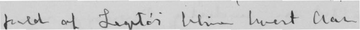fuld af Legetøi blive hvert Aar
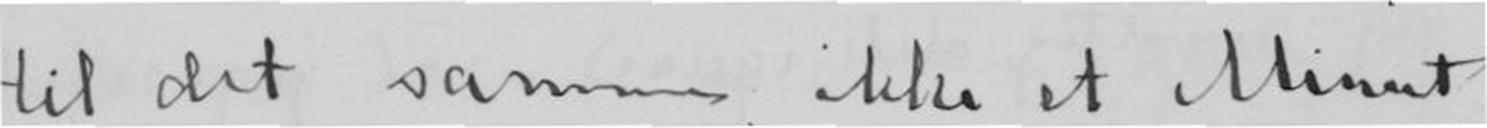til det samme, ikke et Minut
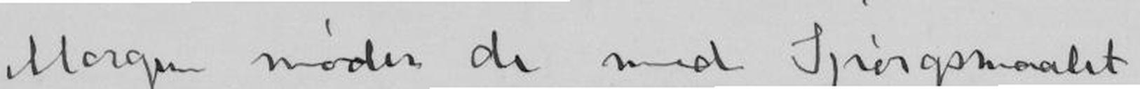Morgen nøder de med Spørsmaalet
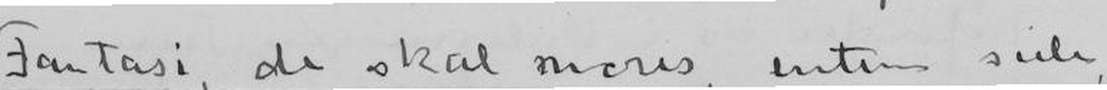Fantasi, de skal mores, enten side,
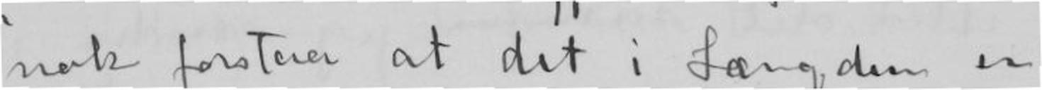nok forstaa at det i Længden er
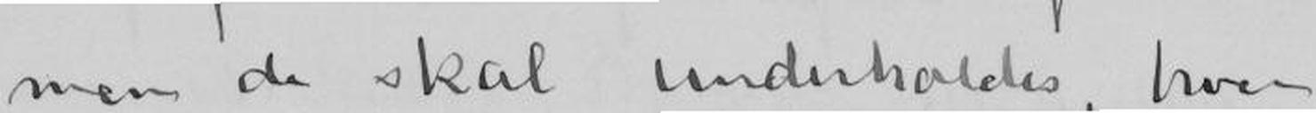men de skal underholdes, hver
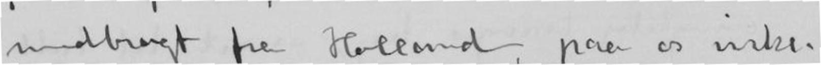medbragt fra Holland, paa os virker
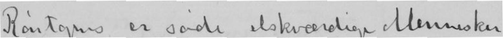Rötgens er søde elskværdige Menneske
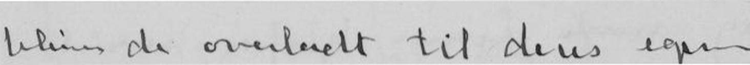blive de overladt til deres egen
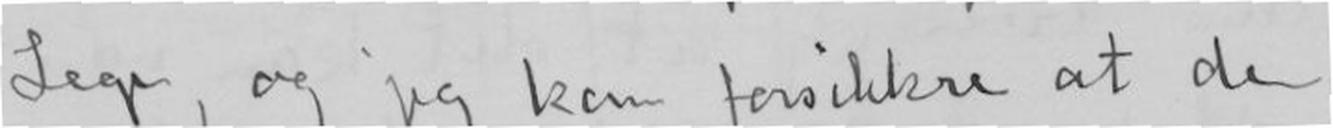Lege, og jeg kan forsikkre at de
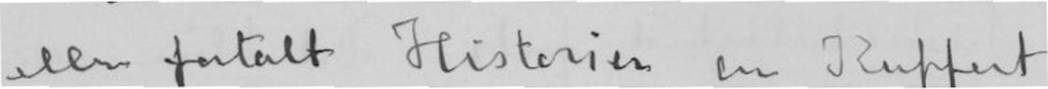eller fortalt Historier, en Kuffert
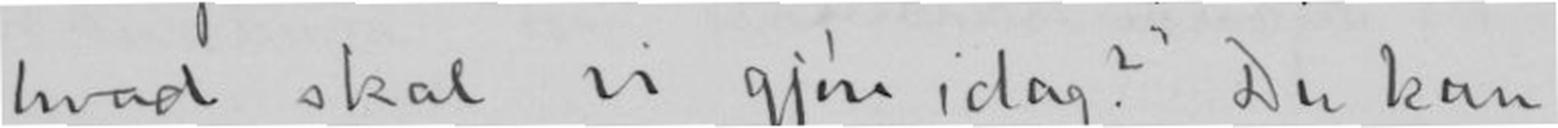"hvad skal vi gjøre idag?" Du kan
